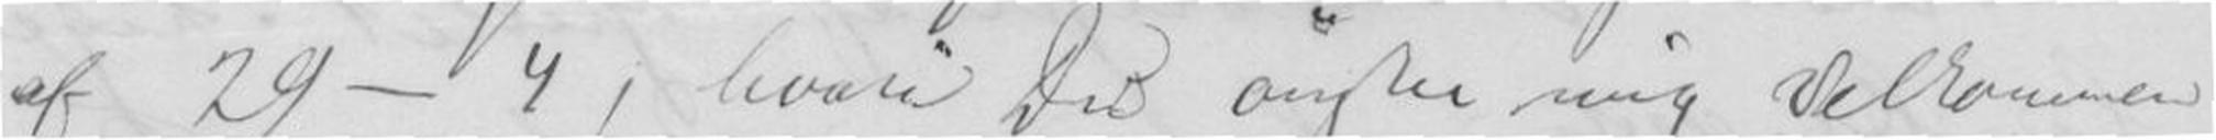af 29-4; hvori Du ønsker mig Velkommen
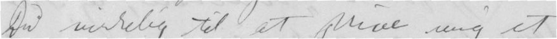Du virkelig til at skrive mig et
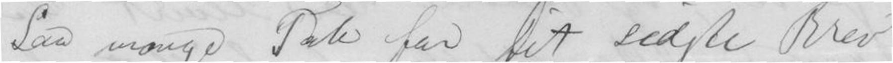Saa mange Tak for Dit sidste Brev
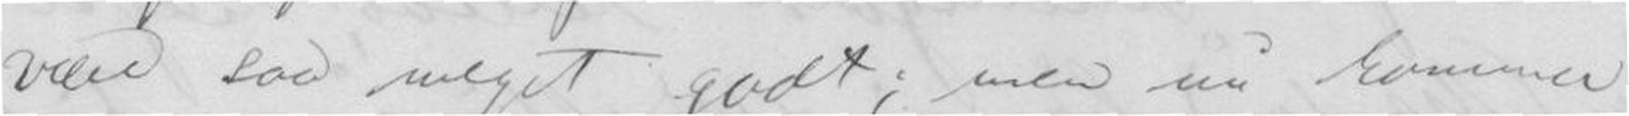være saa meget godt; men nu kommer
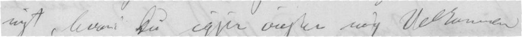nyt, hvori Du igjen ønsker mig Velkommen
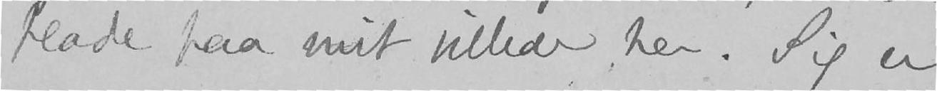plade paa mit billede her. Sig er
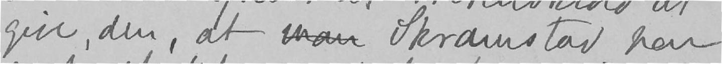give, den, at man Skramstad har
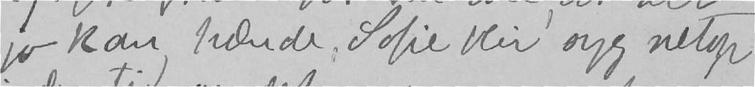jo kan hænde, Sofie blir syg netop
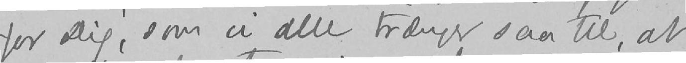for Dig, som vi alle trænger saa til, at
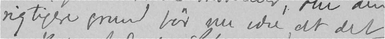rigtigere grund bør nu være, at det
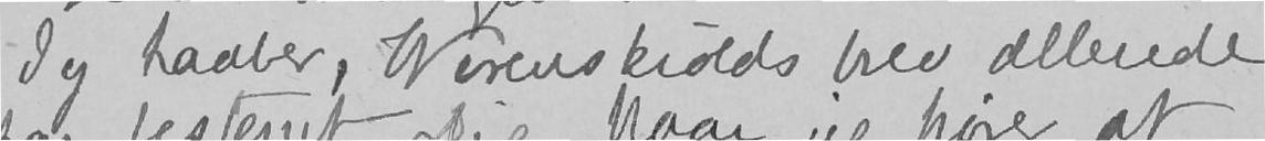Jeg haaber, Werenskiolds brev allerede
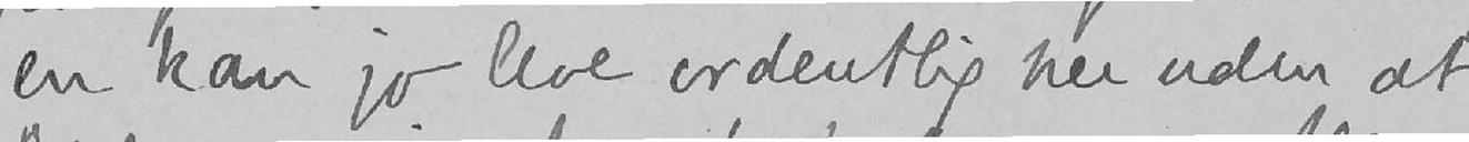en kan jo leve ordentlig her uden at
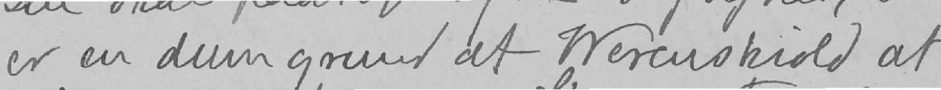er en dum grund af Werenskiold at
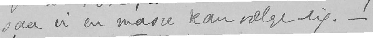saa vi en masse kan vælge Dig. –
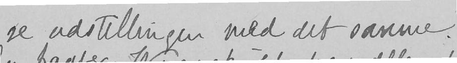se udstillingen med det samme.
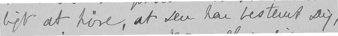ligt at høre, at Du har bestemt Dig,
